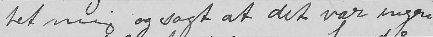tet mig, og sagt at det var ingen
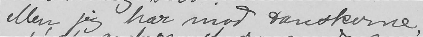Men jeg har mod danskerne,
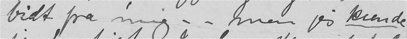bidt fra mig - - men jeg kunde
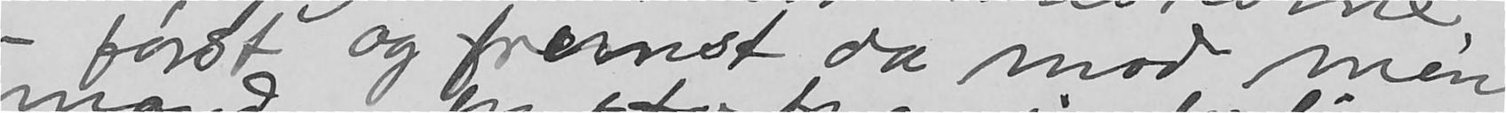- først og fremst da mod min
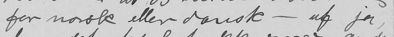for norsk eller dansk - uf ja,
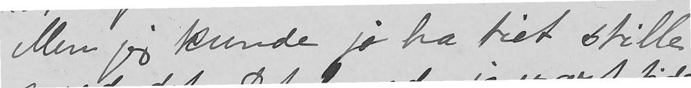Men jeg kunde jo ha tiet stille
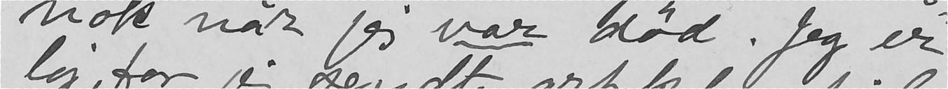nok når jeg var død. Jeg er
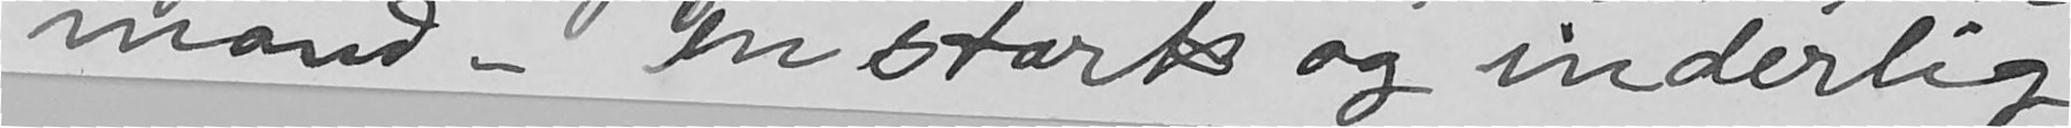mand - en stork og inderlig
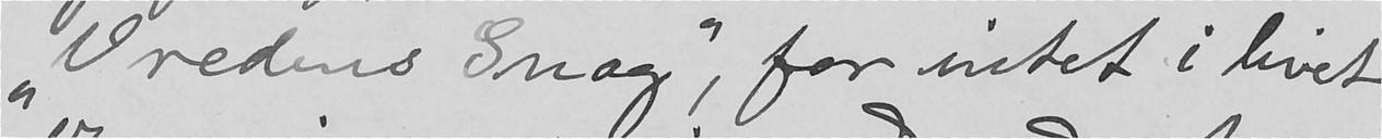"Vredens Gnag", for intet i livet
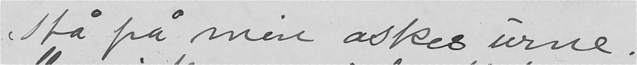stå på min åskes urne.
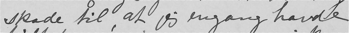skade til, at jeg engang havde
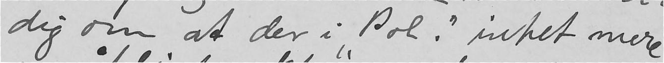dig om at der i "Pol." intet mere
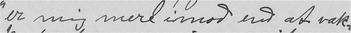er mig mere imod end at væk-
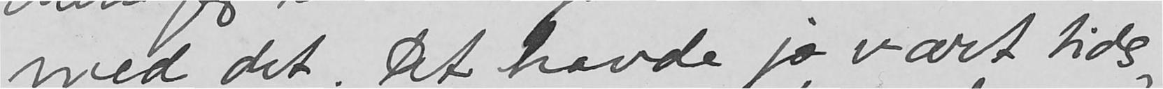med det. Det havde jo vært tids-
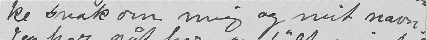ke snak om mig og mit navn.
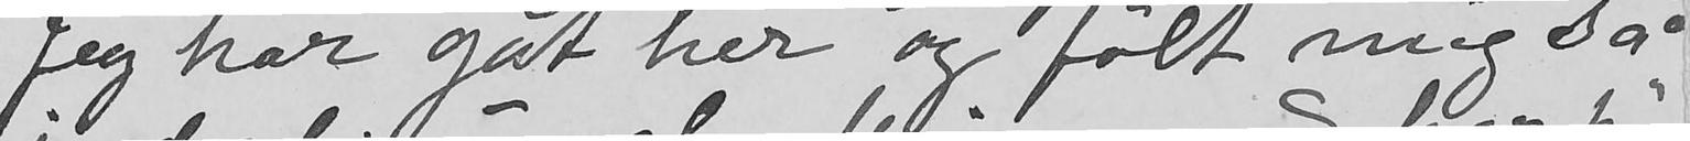Jeg har gåt her og følt mig så
